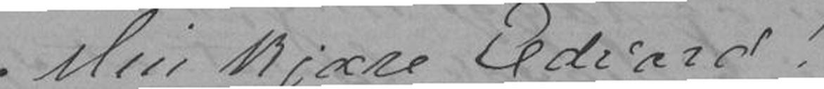Min kjære Edvard!
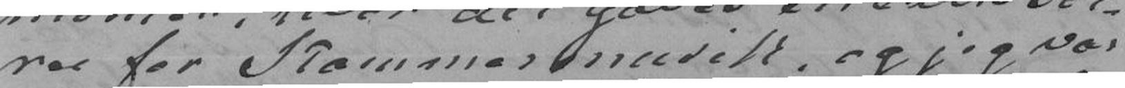ree for Kammermusik, og jeg var
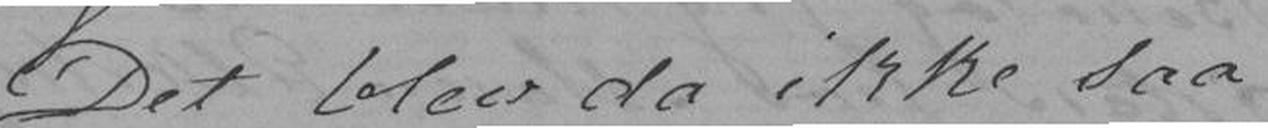Det blev da ikke saa
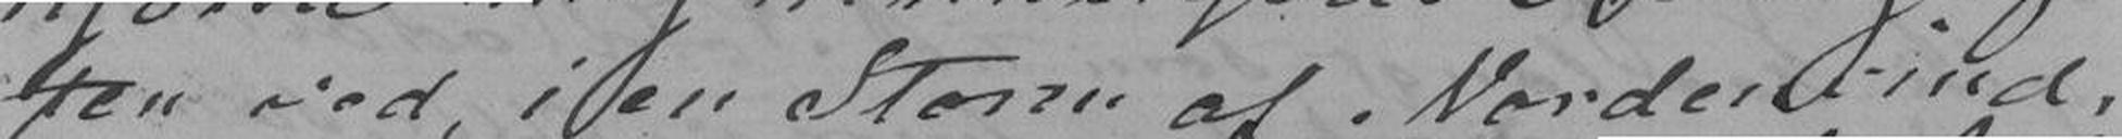ten ved, i en Storm af Nordenvind,
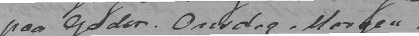paa Gaden. Onsdag Morgen
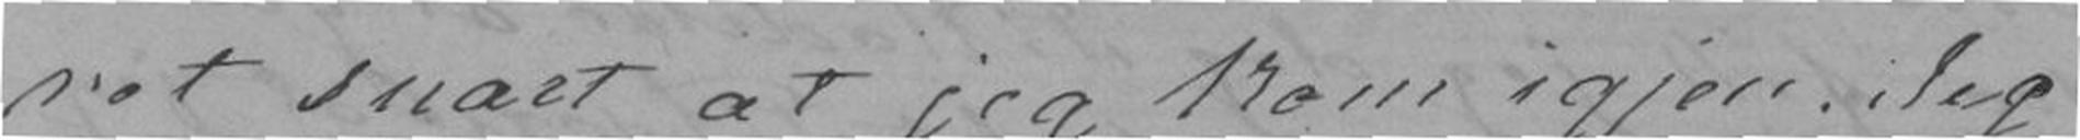ret snart at jeg kom igjen. Jeg
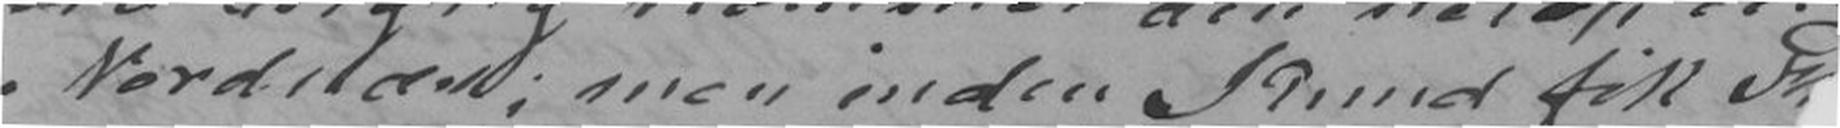Nordnæs, men inden Knud fik
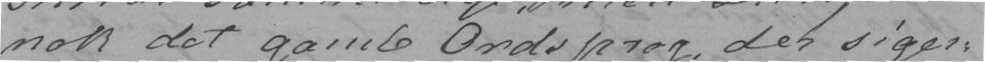nok det gamle Ordsprog der siger
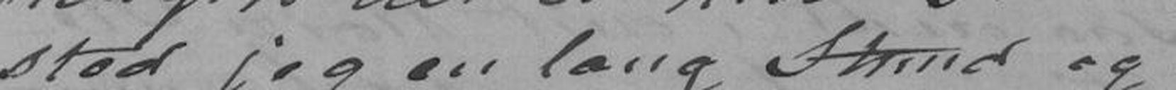stod jeg en lang Stund og
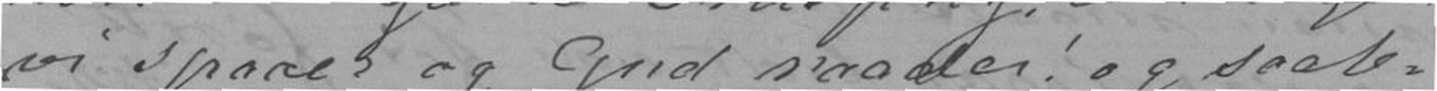vi spaaer og Gud raader! og saale-
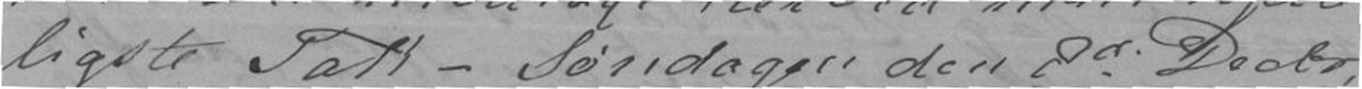ligste Tak - Søndagen den 8d Decbr,
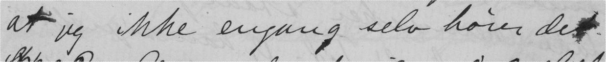at jeg ikke engang selv hører det.
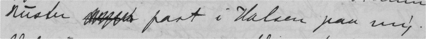ruster  fast i Halsen paa mig.
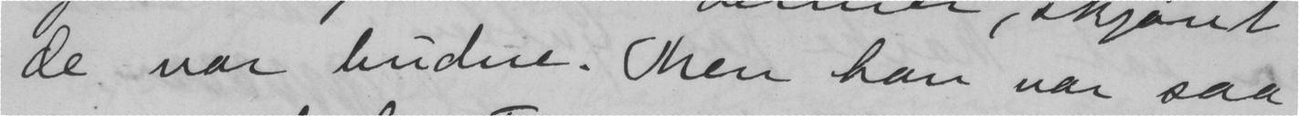de var budne. Men han var saa
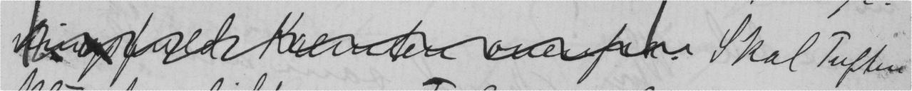Skal Tuften
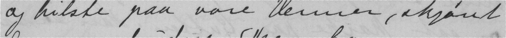og hilste paa vore venner, skjønt
